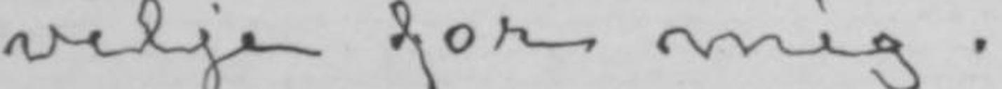vilje for mig.
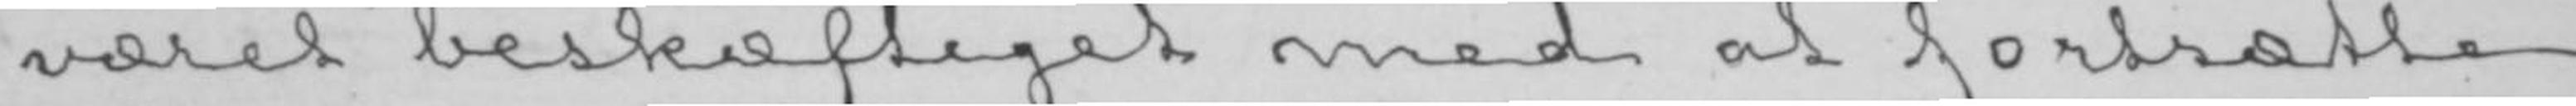været beskæftiget med at fortsætte
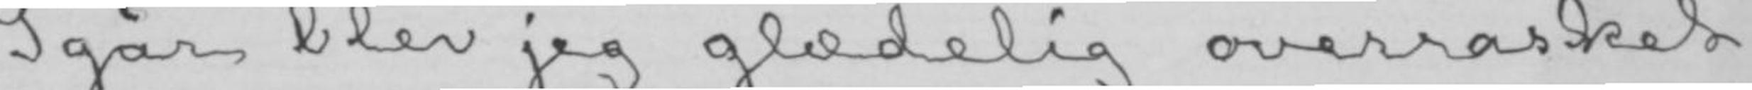Igår blev jeg glædelig overrasket
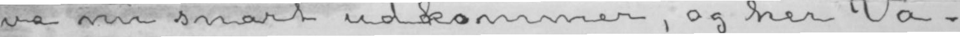ve nu snart udkommer, og her Va-
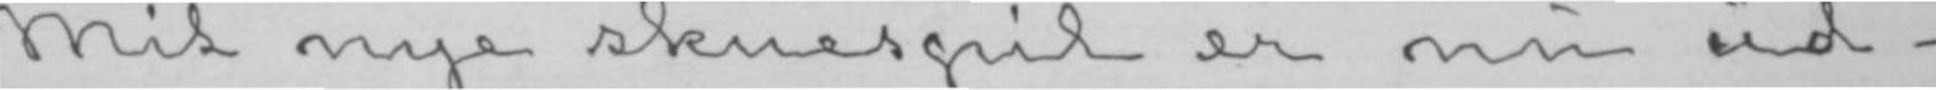Mit nye skuespil er nu ud-
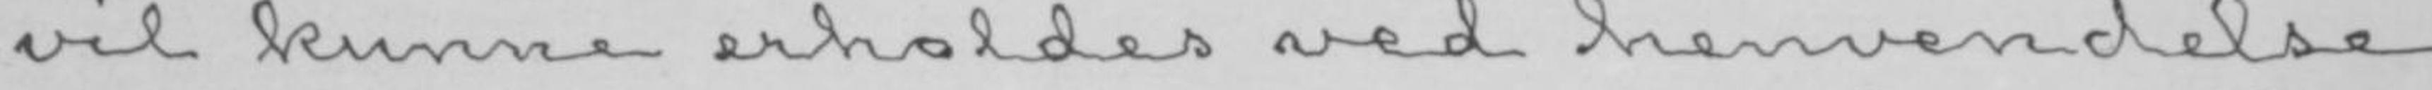vil kunne erholdes ved henvendelse
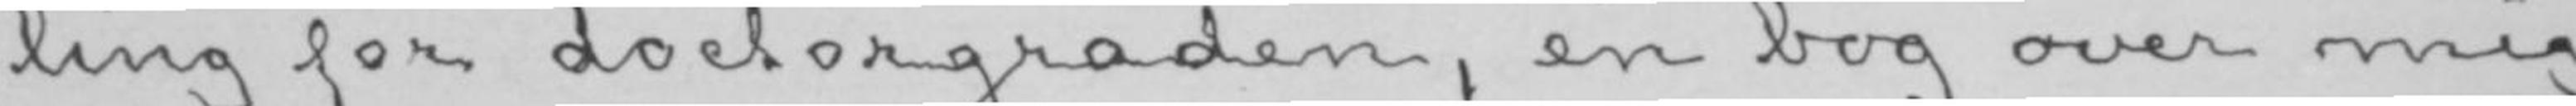ling for doctorgraden, en bog over mig
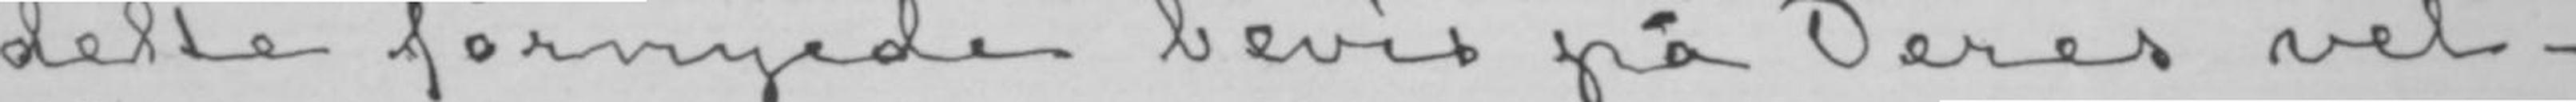dette fornyede bevis på Deres vel-
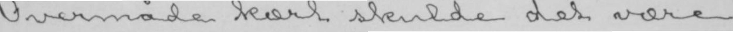Overmåde kært skulde det være
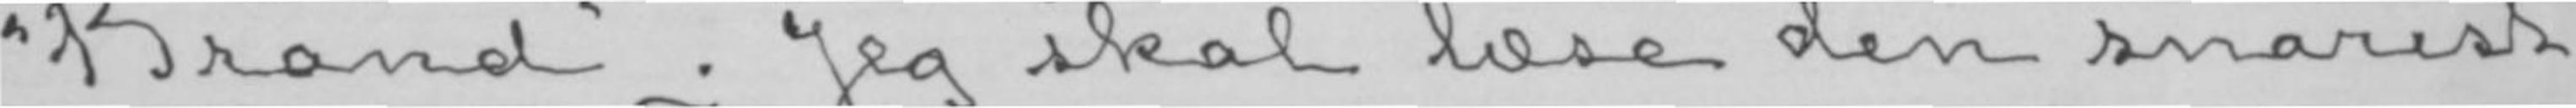«Brand». Jeg skal læse den snarest
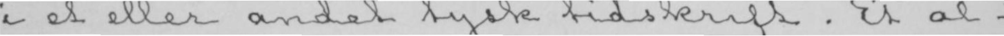i et eller andet tysk tidskrift. Et al-
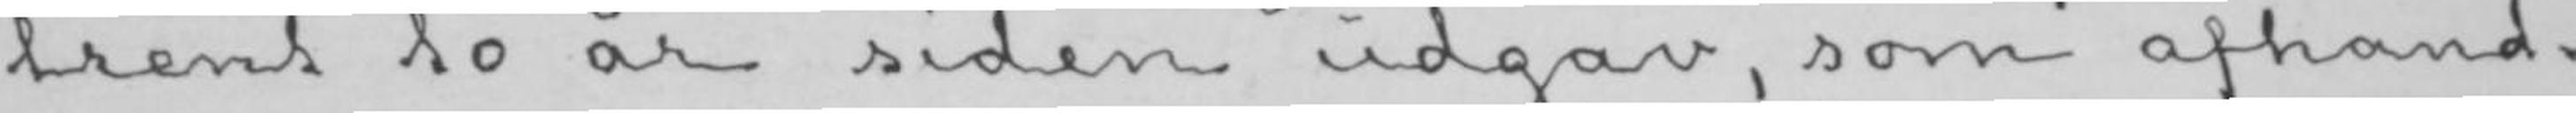trent to år siden udgav, som afhand-
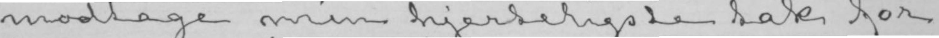modtage min hjerteligste tak for
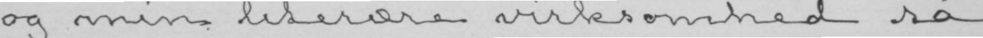og min literære virksomhed så
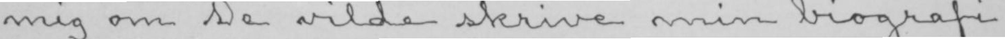mig om De vilde skrive min biografi
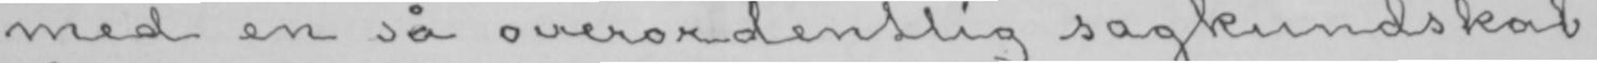med en så overordentlig sagkundskab
